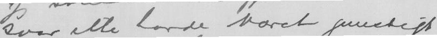svar ikke havde været gunstigt.
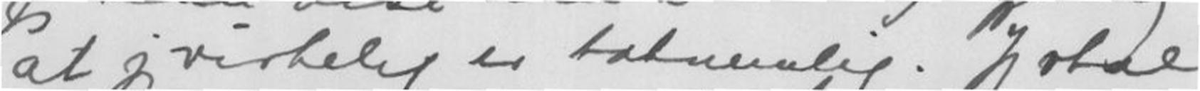at jeg virkelig er taknemlig. Jeg skal
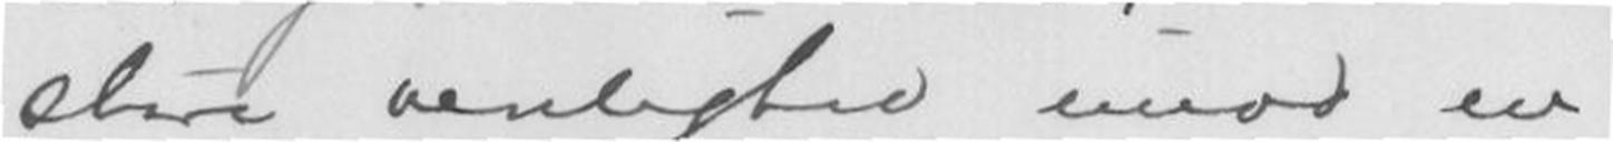store venlighed mod en
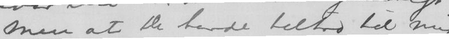Men at De havde tiltro til mig
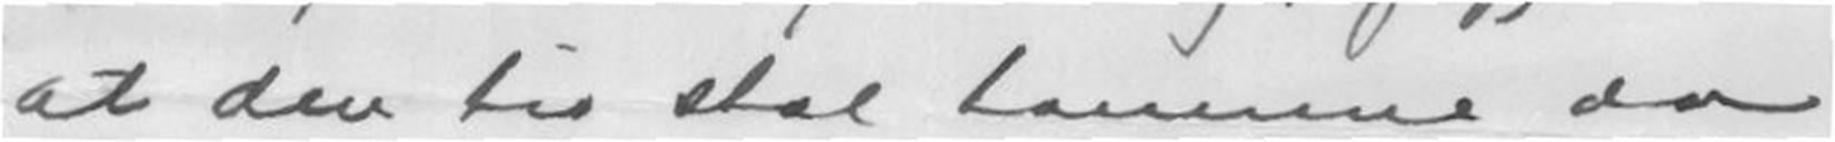at den tid skal komme da
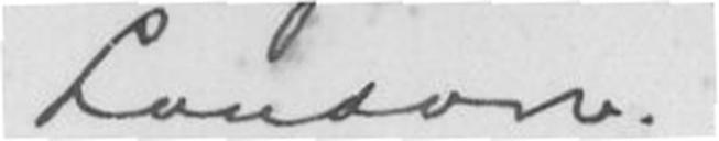London W.
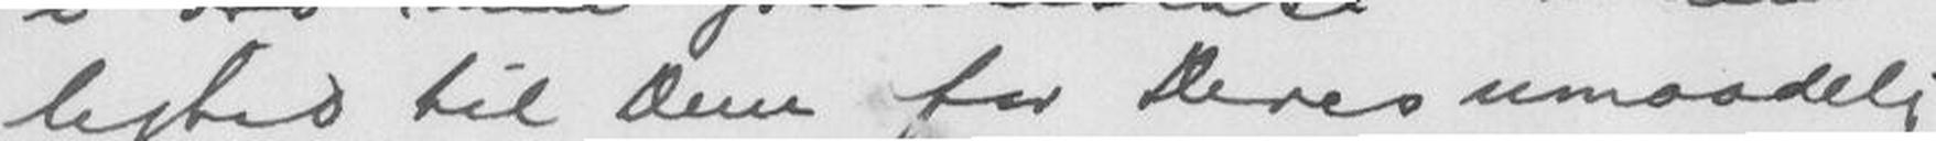lighed til Dem for Deres umaadelig
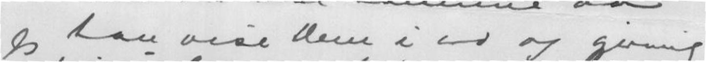jeg faar vise Dem i ord og gjerning
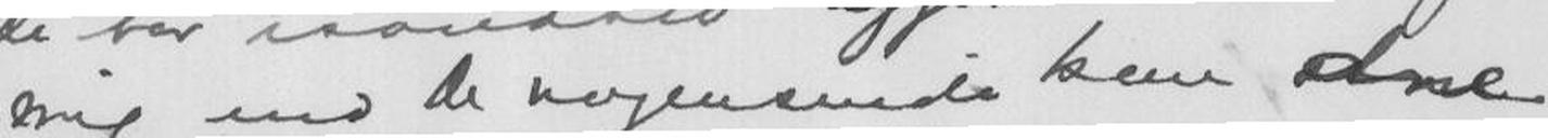mig end De nogensinde kan ane
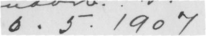6.5.1907
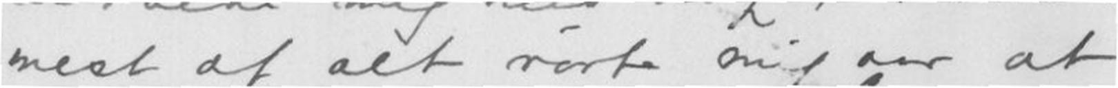mest af alt rørte mig var at
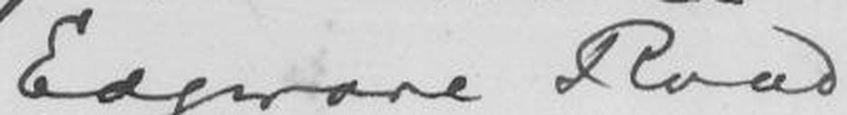Edgmare Rood
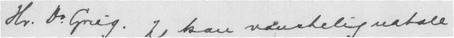Hr. Dr Grieg. Jeg kan vanskelig udtale
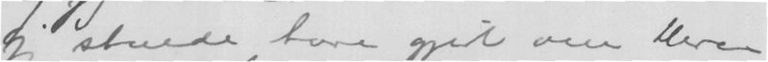jeg skulde have gjort om Deres
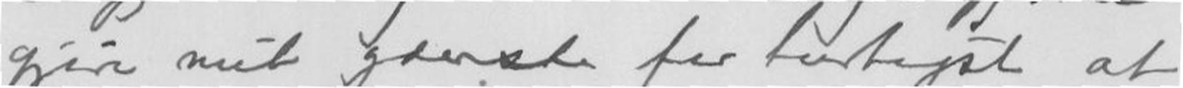gjøre mit yderste for hurtigst at
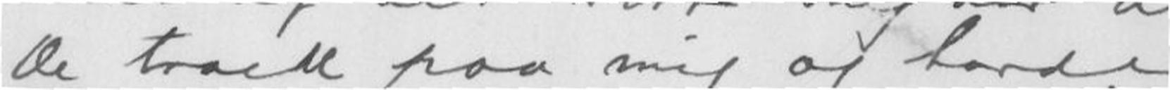De troede paa mig og havde
